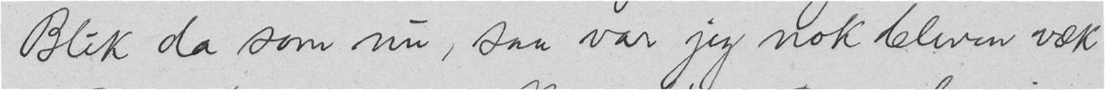Blik da som nu, saa var jeg nok bleven væk
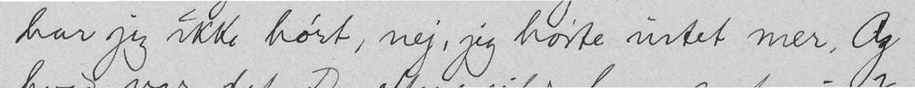har jeg ikke hørt, nej, jeg hørte intet mer. Og
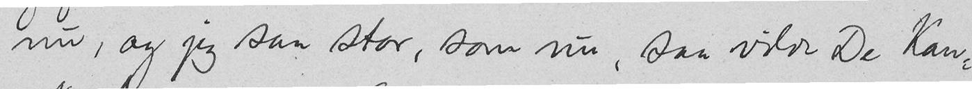nu, og jeg saa stor, som nu, saa vilde De kan-
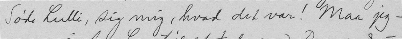Søde Lulli, sig mig, hvad det var! Maa jeg -
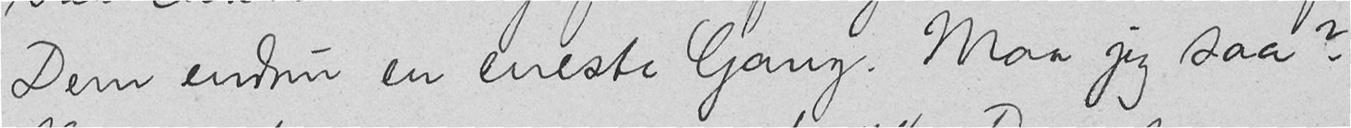Dem endnu en eneste Gang. Maa jeg saa?
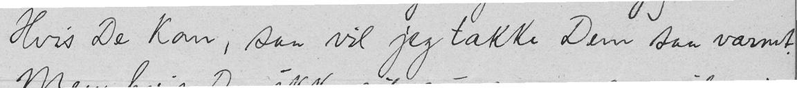Hvis De kan, saa vil jeg takke Dem saa varmt.
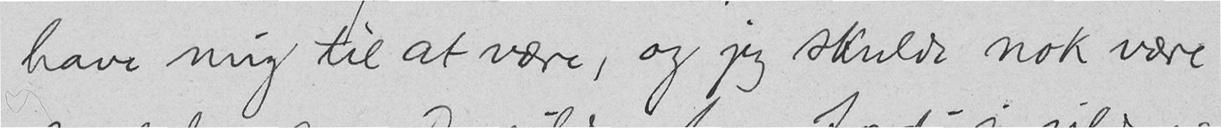have mig til at være, og jeg skulde nok være
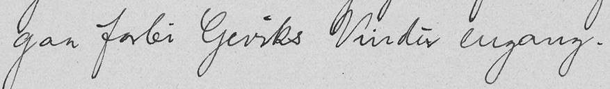gaa forbi Geviks Vindu engang.
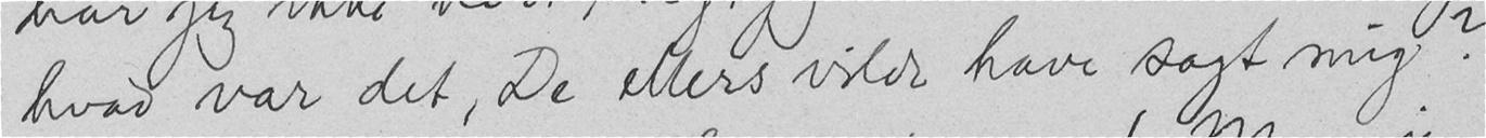hvad var det, De ellers vilde have sagt mig?
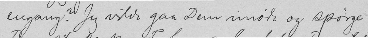engang? Jeg vilde gaa Dem imøde og spørge
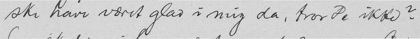ske have været glad i mig da, tror De ikke?
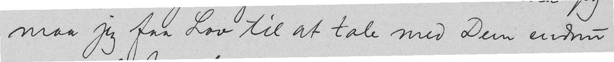maa jeg faa Lov til at tale med Dem endnu
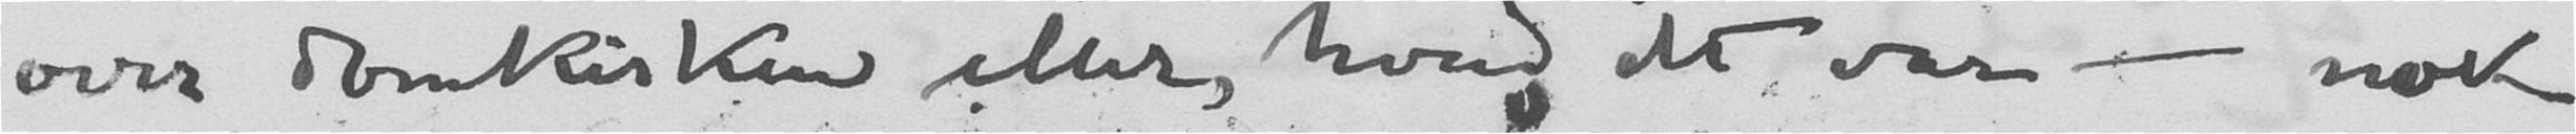over domkirken eller, hvad det var - nok
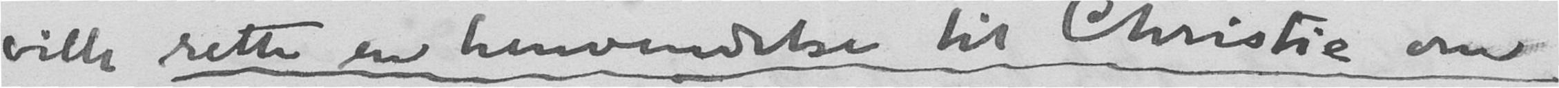ville sette en henvendelse til Christie om
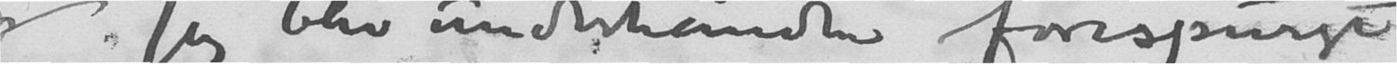Jeg blev underhånden forespurgt
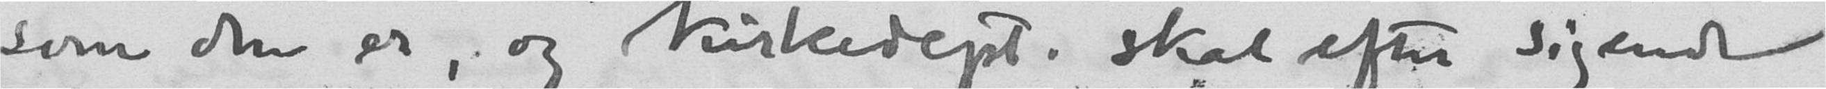som den er, og kirkedept. skal efter sigende
